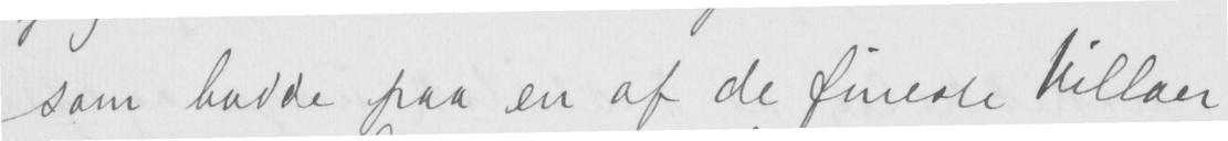som bodde paa en af de fineste Villaer
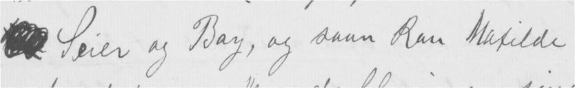Seier og Bay, og saan kan Matilde
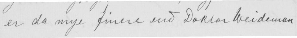er da mye finere end Doktor Weideman
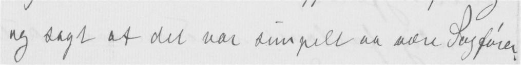og sagt at det var simpelt aa være Sagfører.
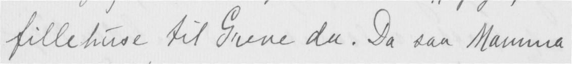fillehuse til Greve da. Da saa Mamma
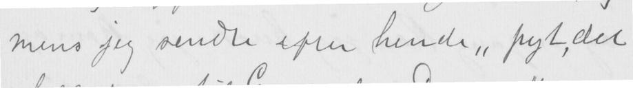mens jeg sendte efter hende "pyt, det
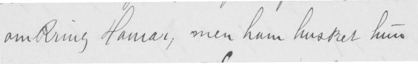omkring Hamar; men ham husket hun
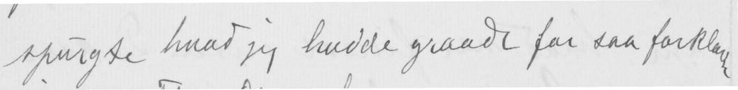spurgte hvad jeg hadde graadt for saa forklarte
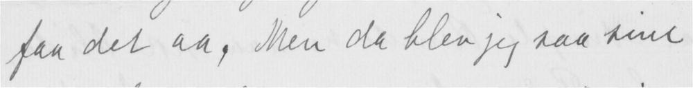faa det aa. Men da blev jeg saa sint
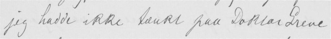jeg hadde ikke tænkt paa Doktor Greve
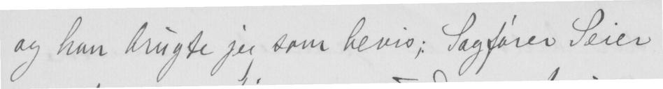og han brugte jeg som bevis; Sagfører Seier
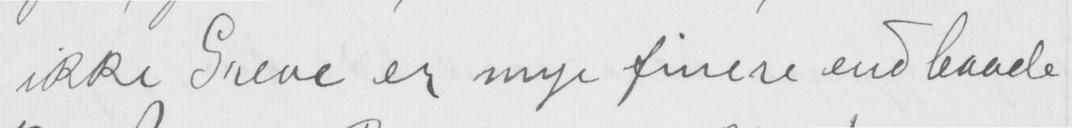ikke Greve er mye finere end baade
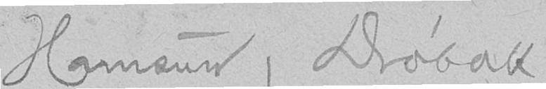Hamsun, Drøbak
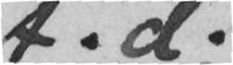t.d.
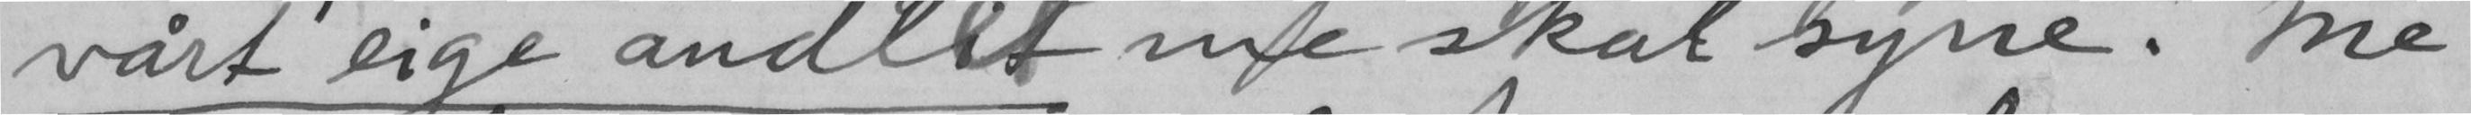vårt eige andlet me skal syne. Me
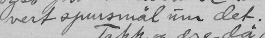vert spursmål um det.
